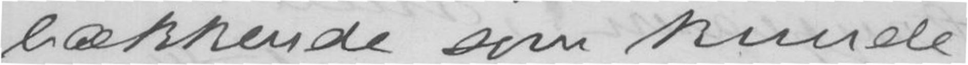bækkende, som kunde
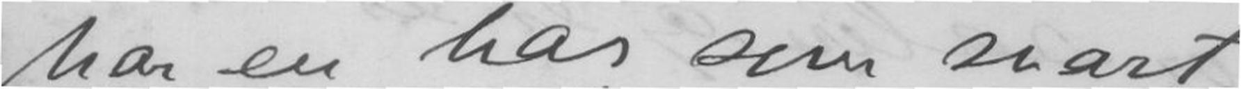har en bas som svart
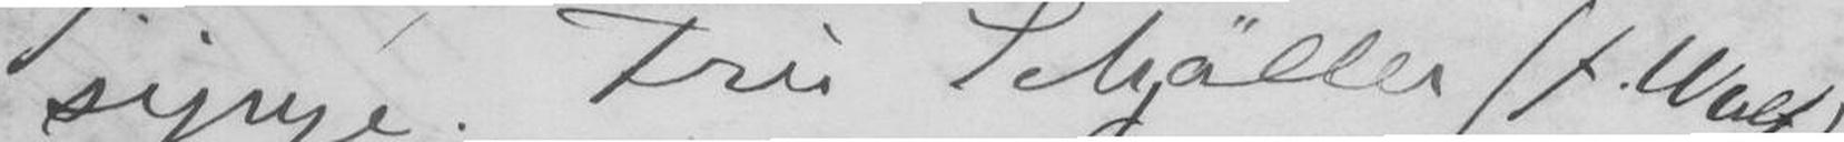synge. Fru Schjøller (f. Wolf).
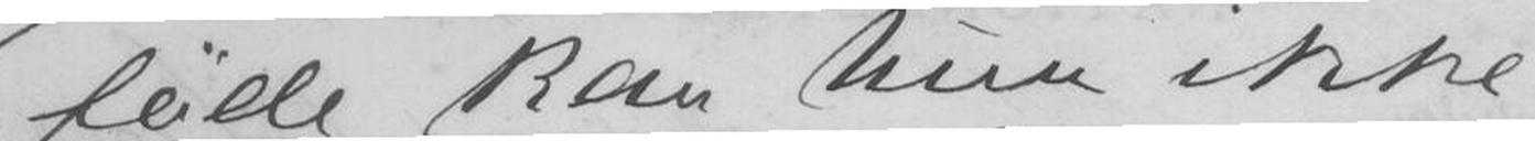føde, kan hun ikke
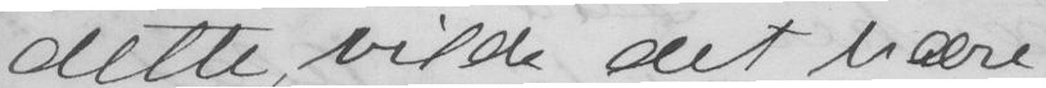dette, vilde det være
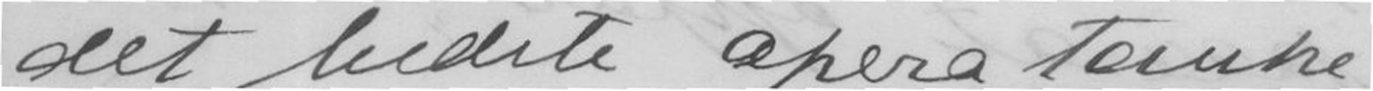det bedste opera tanke
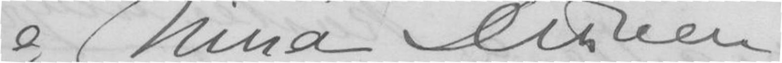og Nina Din ven
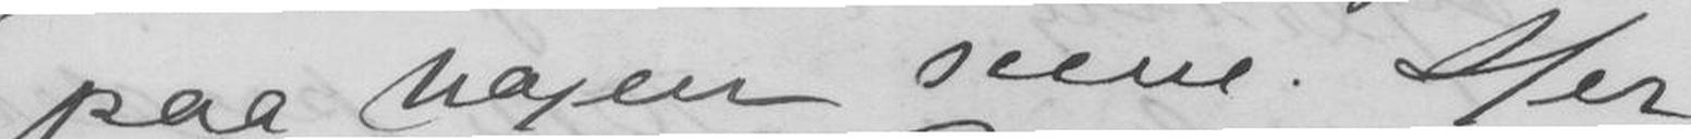paa nogen scene. Her
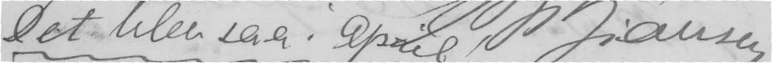Det blev saa i April! B. Bjørnson
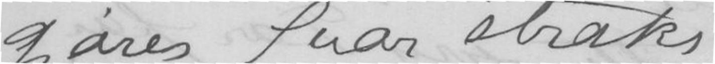gjøres Svar straks.
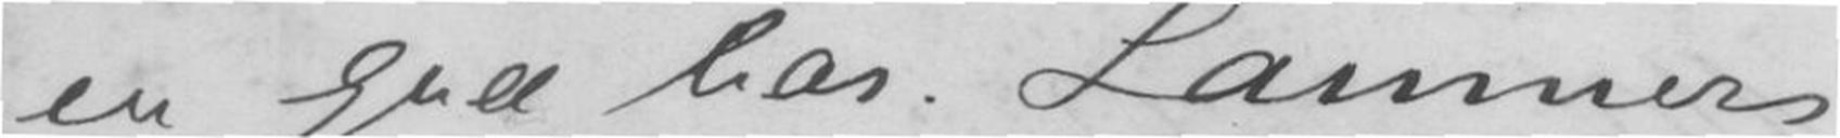en god bas. Lammers
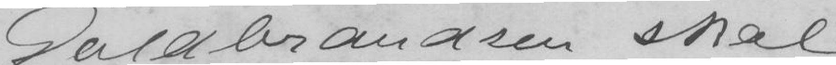Guldbrandsen skal
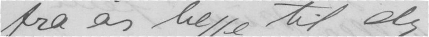fra os begge til dig
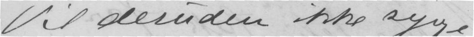Vil desuden ikke synge
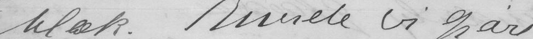blæk. Kunde vi gjøre
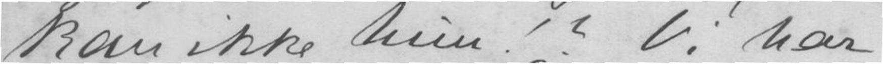Kan ikke hun!? Vi har
